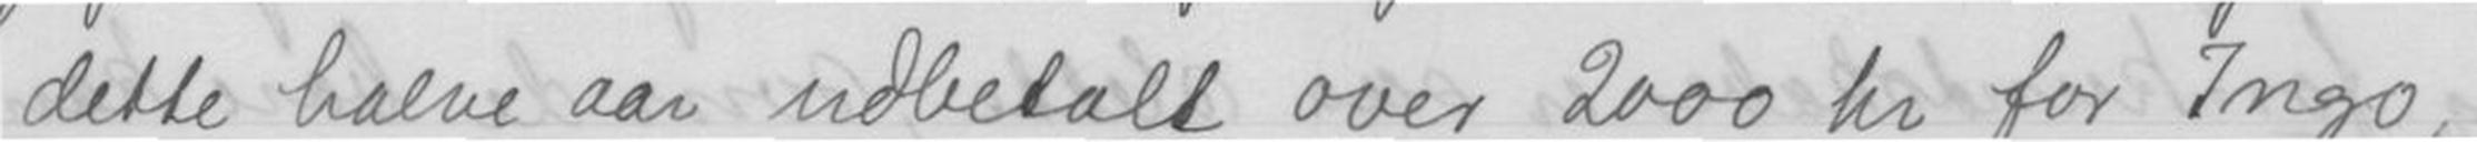dette halve aar udbetalt over 2000 kr for Ingo,
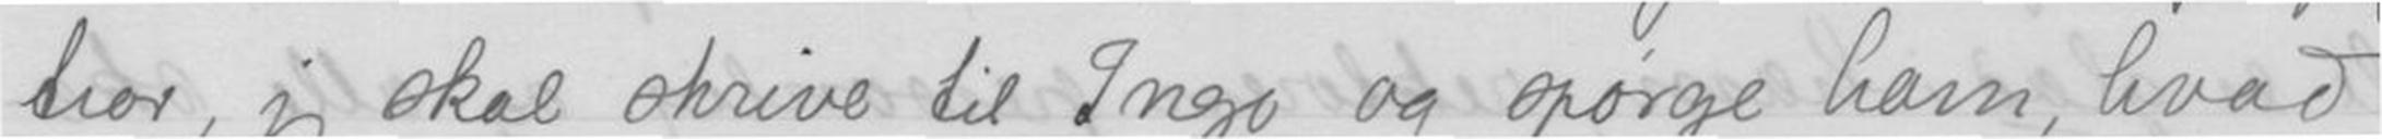tror, jeg skal skrive til Ingo og spørge ham, hvad
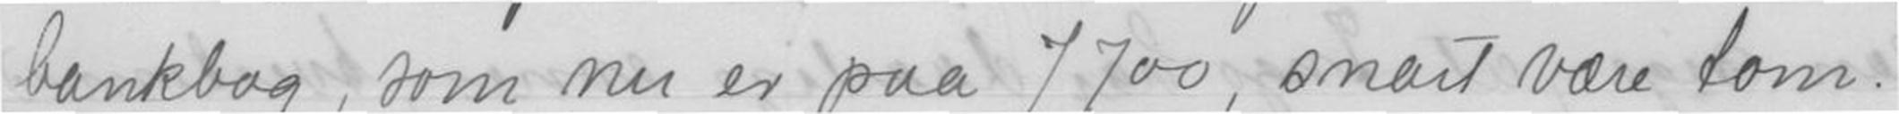bankbog, som nu er paa 7 700, snart være tom.
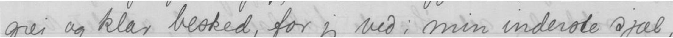grei og klar besked, for jeg ved i min inderste sjæl,
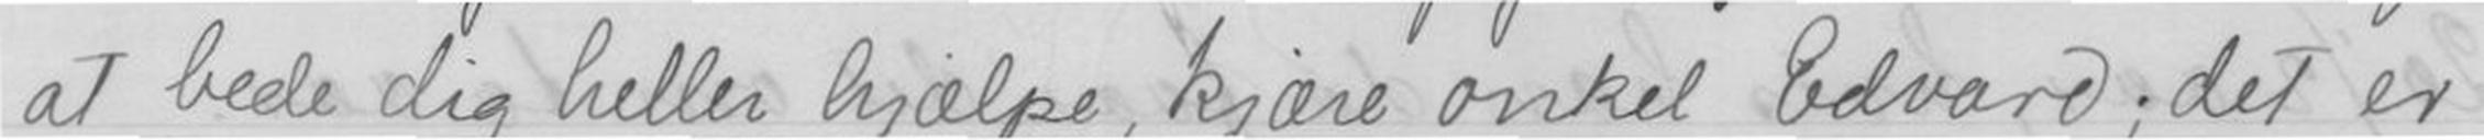at bede dig heller hjælpe, kjære onkel Edvard; det er
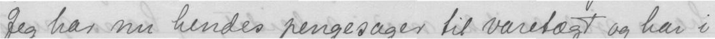Jeg har nu hendes pengesager til varetægt og har i
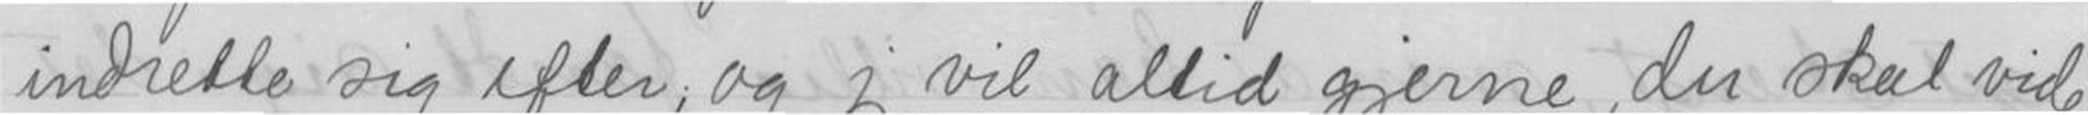indrette sig efter; og jeg vil altid gjerne, du skal vide
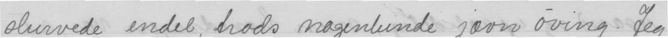slurvede endel, trods nogenlunde jævn øving. Jeg
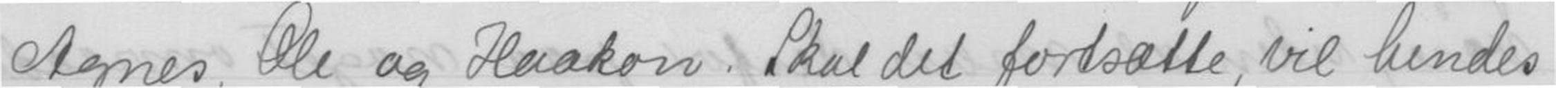Agnes. Ole og Haakon. Skal det fortsætte, vil hendes
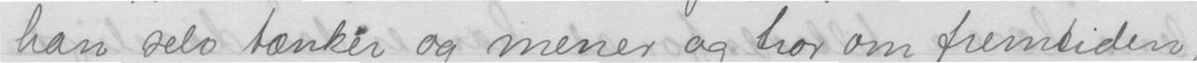han selv tænker og mener og tror om fremtiden,
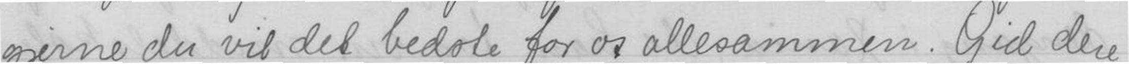gjerne du vil det bedste for os allesammen. Gid dere
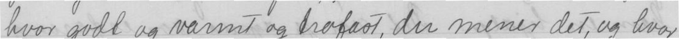hvor godt og varmt og trofast, du mener det, og hvor
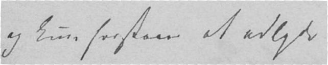og kun forstaaer at adlyde
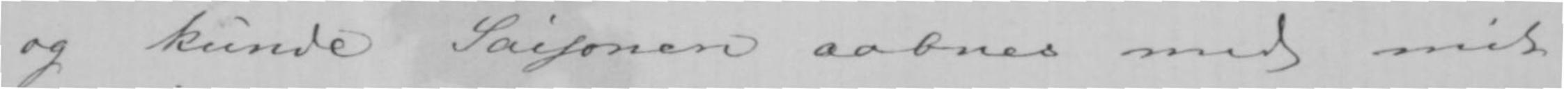og kunde Saisonen aabnes med mit
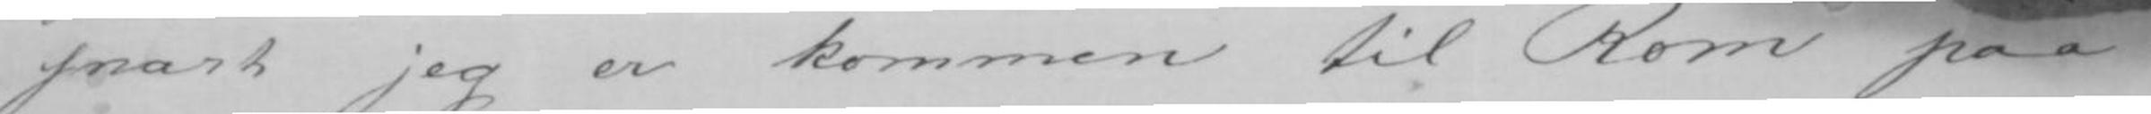snart jeg er kommen til Rom paa-
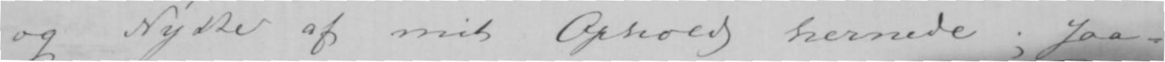og Nytte af mit Ophold hernede; saa-
